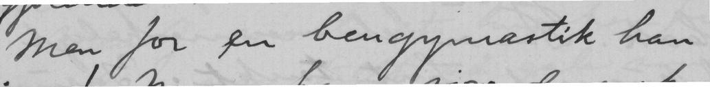Men for en bengymnastik han
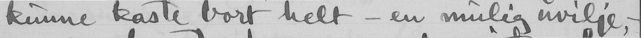kunne kaste bort helt - en mulig uvilje, -
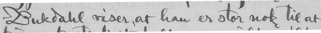Bukdahl viser, at han er stor nok til at
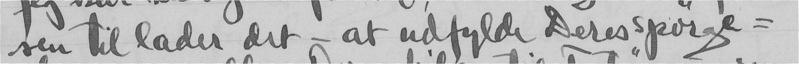sen til lader det - at udfylde Deres spørge-
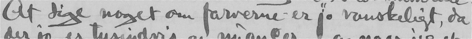At sige noget om farverne er jo vanskeligt, da
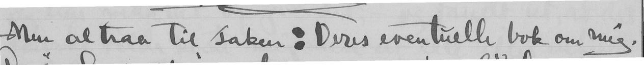Men altsaa til saken ; Deres eventuelle bok om mig.
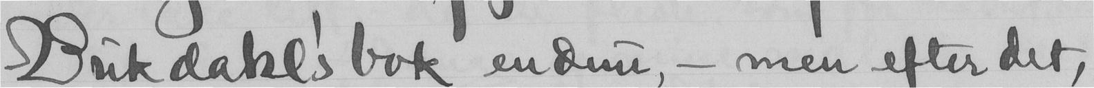Bukdahl's bok endnu, - men efter det,
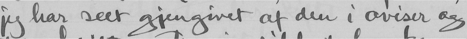jeg har seet gjengivet af den i aviser og
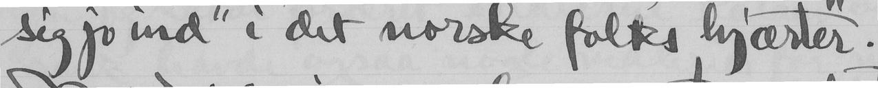sig jo ind" i det norske folks hjærter.
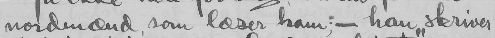nordmænd som læser ham: - han "skriver
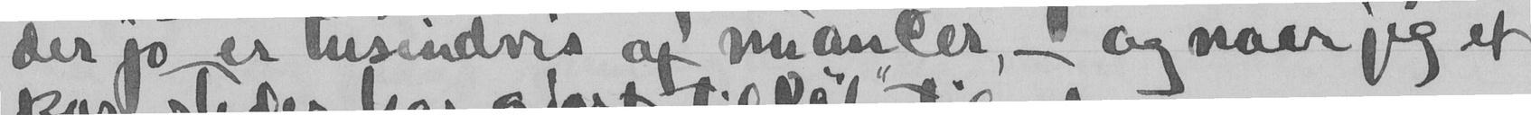der jo er tusendvis af nuancer, - og naar jeg et
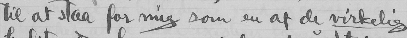til at staa for mig som en af de virkelig
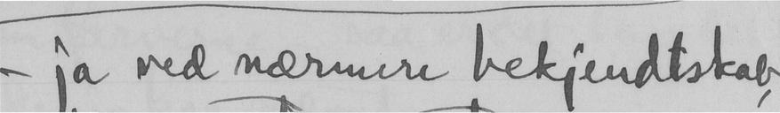- ja ved nærmere bekjendtskab,
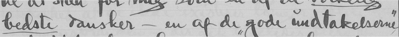bedste dansker - en af de "gode undtakelserne"
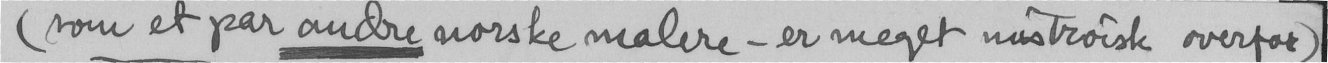(som et par andre norske malere - er meget mistroisk overfor)
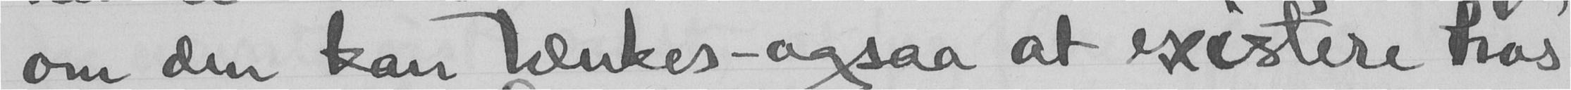om den kan tænkes - ogsaa at existere hos
